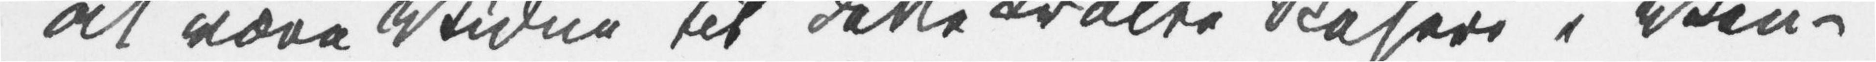at være Vidne til dette kvalte Raseri i Ven¬
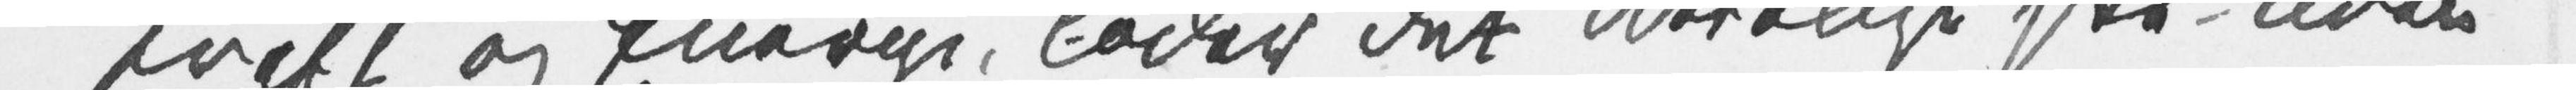Kraft og Energi, lader det Utrolige ske – uden
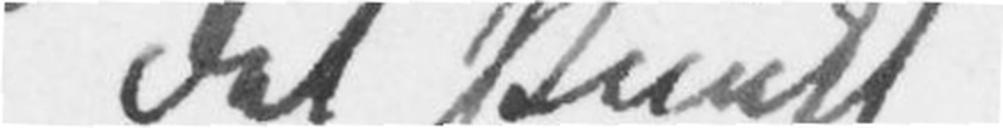det Punkt
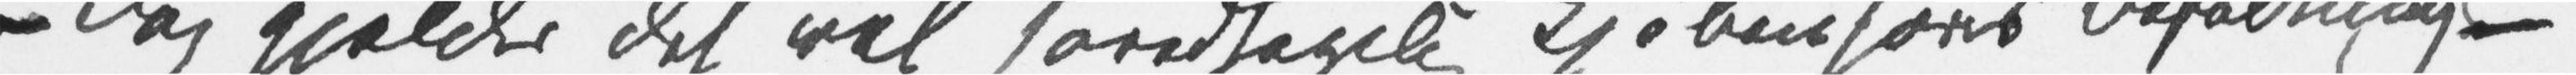– dog gjelder det vel hovedsagelig Kjøbenhavns Befolkning –
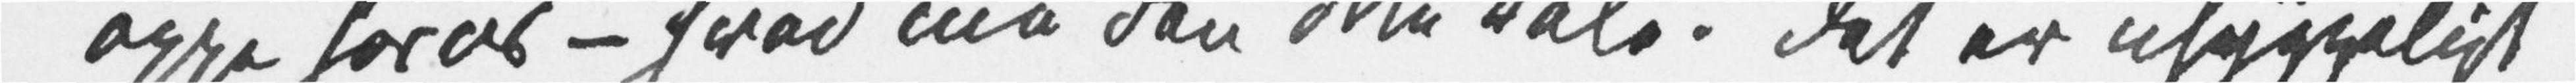oppe hos os – hvad må den ikke tåle! Det er uhyggeligt
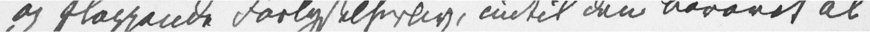og flagrende Forlystelsesliv, indtil den berøvet al
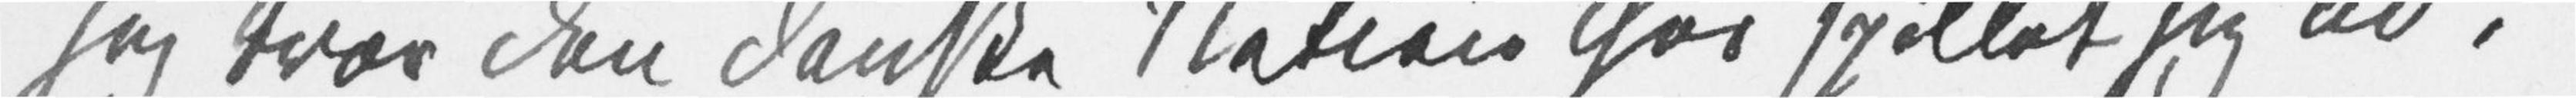Jeg tror den danske Nation har spillet sig ud,
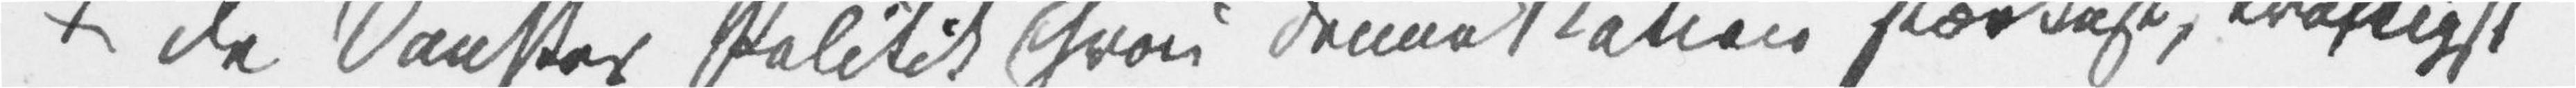i de Danskes Politik hvori denne Nation stærkest, kraftigst
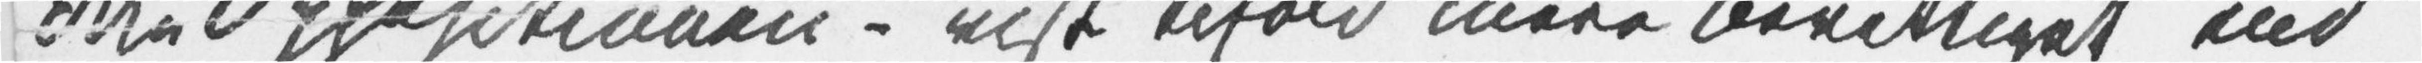ikke Oppositionen – vist tifold mere berettiget end
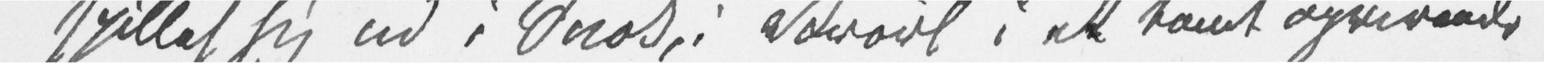spillet sig ud i Snak, i Vrøvl i et tomt oprivende
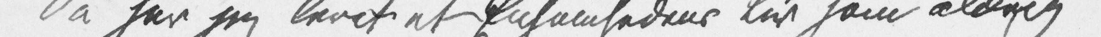Så har jeg levet et Ensomhedens Liv som aldrig
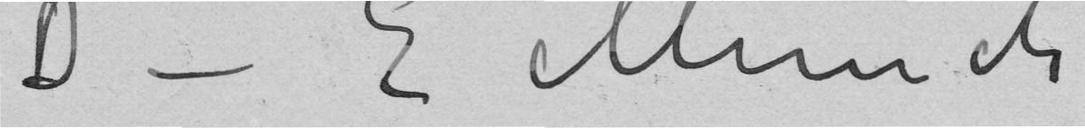Din E Munch
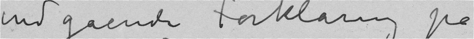indgående Forklaring på
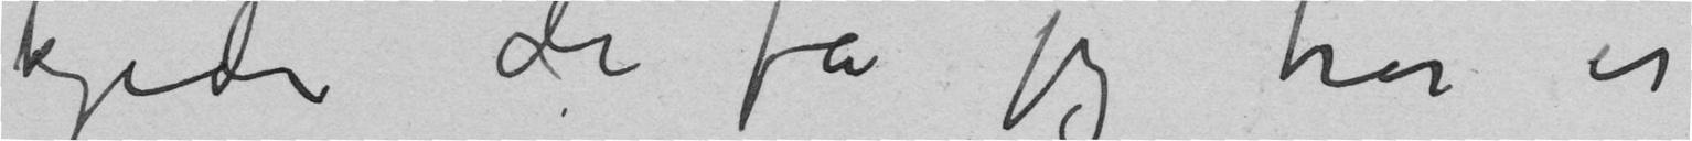kjede de få jeg tror er
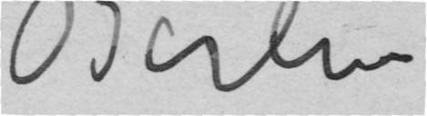Berlin
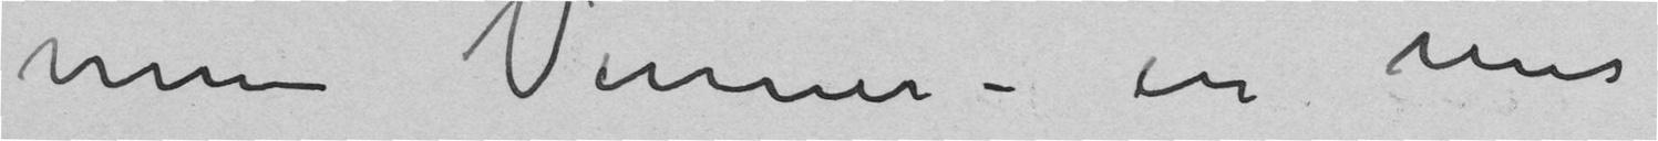mine Venner – en mer
